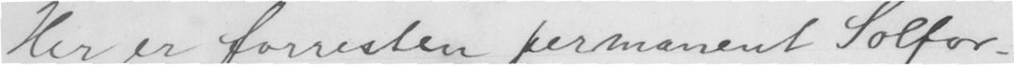Her er forresten permanent Solfor-
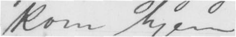kom hjem.
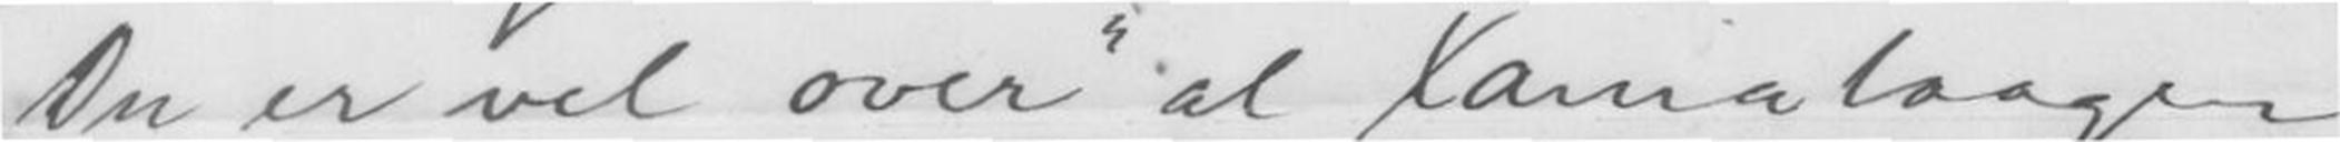Du er vel over al Xaniataagen
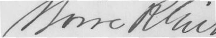Børre R Giertsen
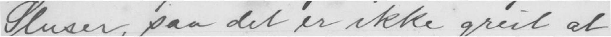Sluser, saa det er ikke greit at
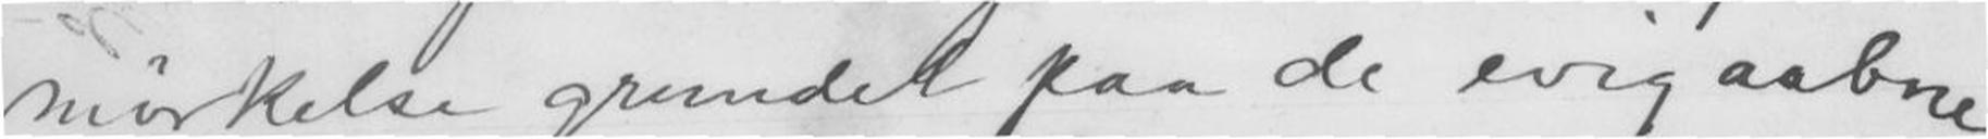mørkelse grundet paa de evig aabne
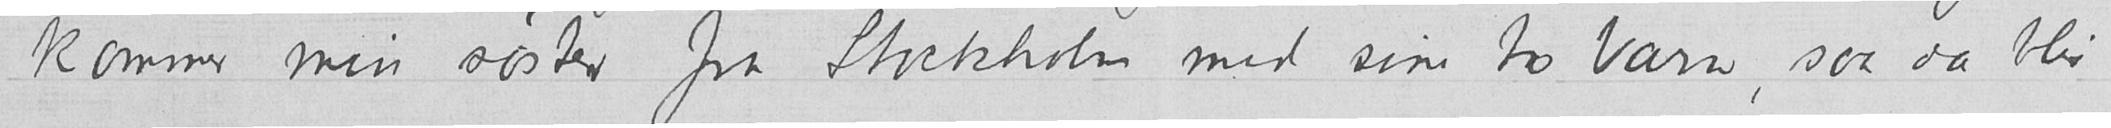kommer min søster fra Stockholm med sine to barn, saa da blir
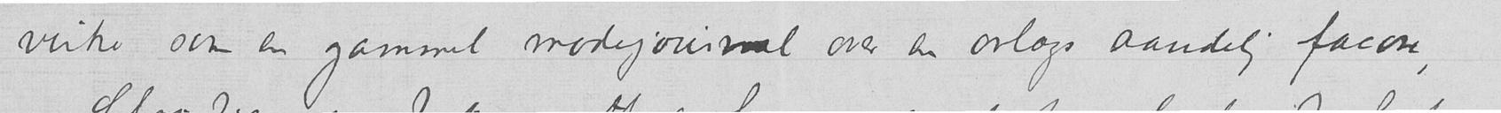virke som en gammel modejournal over en avlægs aandelig facon,
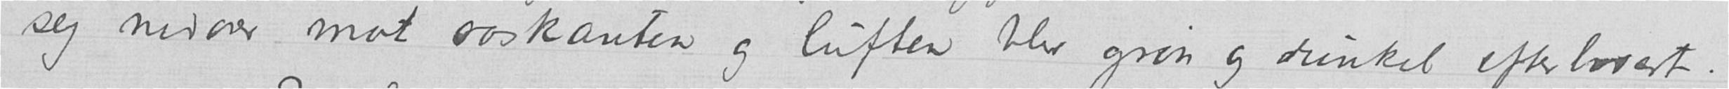seg nedover mot aaskanten og luften blev grøn og dunkel efterhvert.
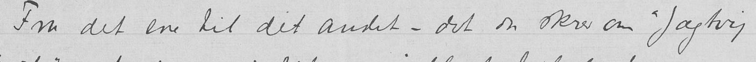Fra det ene til det andet – det du skrev om "Jægtvig
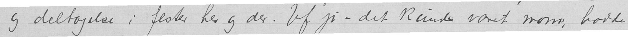og deltagelse i fester her og der. Uf jo – det kunde været morro, hadde
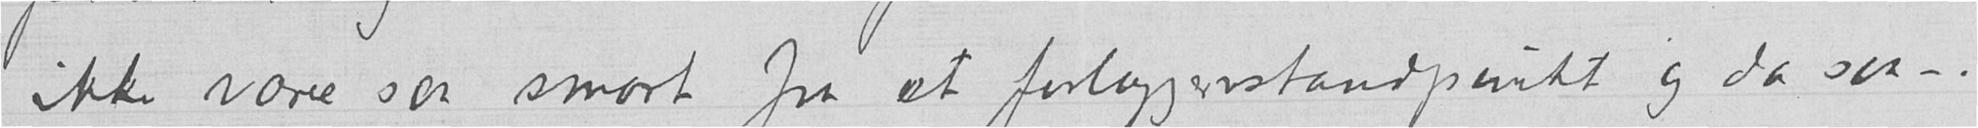ikke være saa smart fra et forlæggerstandpunkt og da saa –.
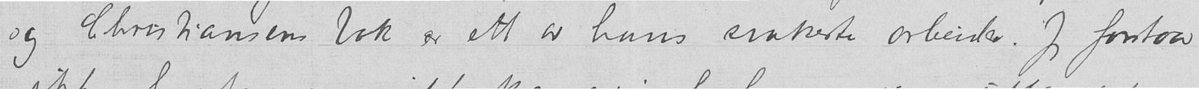og Christiansens bok er ett av hans svakeste arbeider. Jeg forstaar
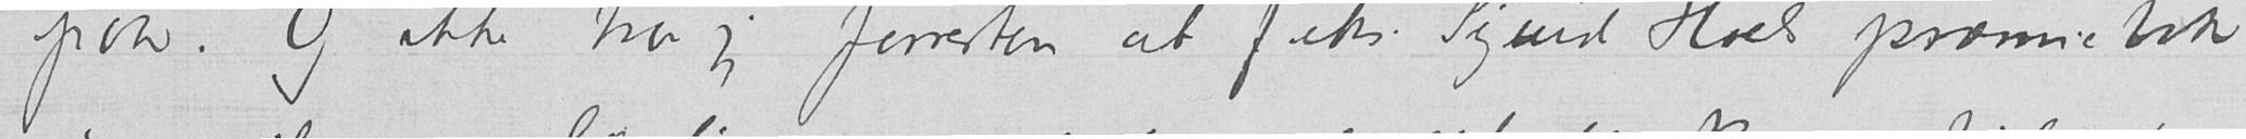paa. Og ikke tror jeg forresten at feks. Sigurd Hoels præmiebok
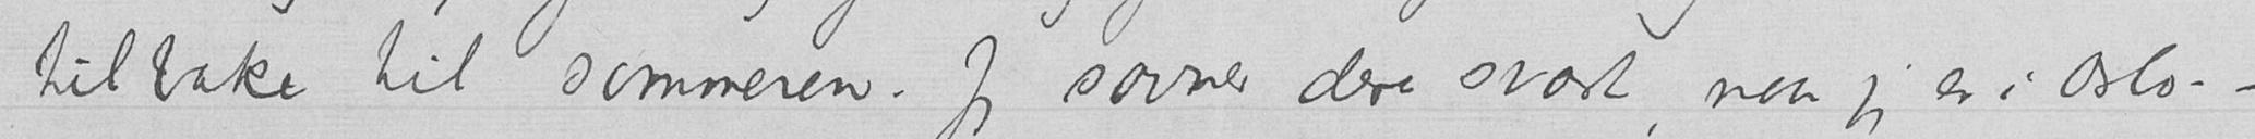tilbake til sommeren. Jeg savner dere svært, naar jeg er i Oslo. –-
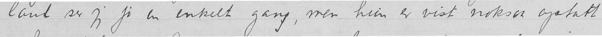land ser jeg jo en enkelt gang, men hun er vist noksaa optatt
